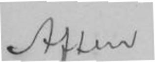Aften
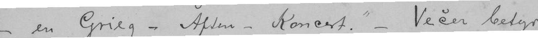- en Grieg - Aften - Koncert. - Veier betyr
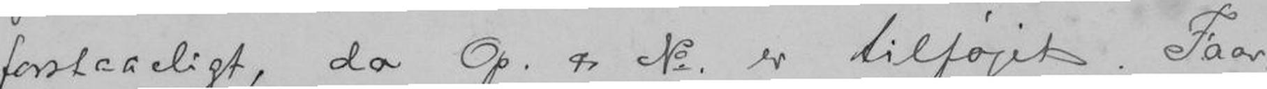forstaaeligt, da Op. & No er tilføjet. Faar
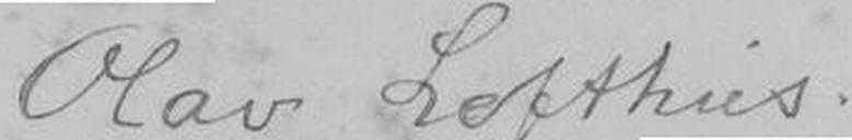Olav Lofthus.
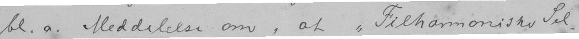bl. a. Meddelelse om, at Filharmoniske Sel-
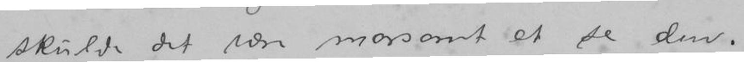skulde det være morsomt at se den.
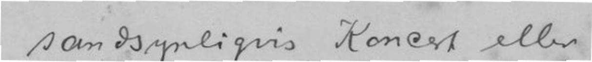sandsynligvis Koncert eller
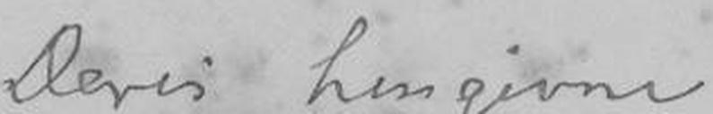Deres hengivne
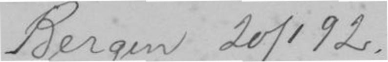Bergen 20/1 92.
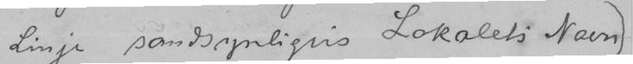Linje sandsynligvis Lokalets Navn)
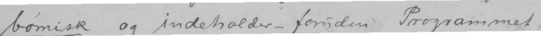bømisk og indeholder - foruden Programmet -
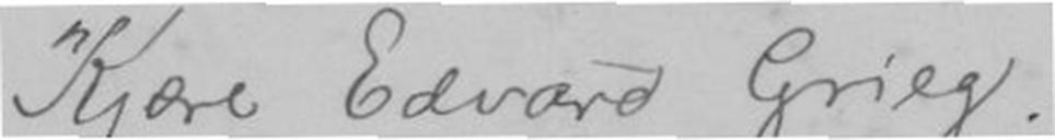Kjære Edvard Grieg.
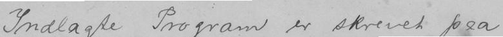Indlagte Program er skrevet paa
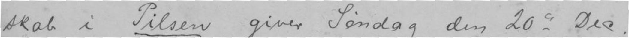skab i Pilsen giver Søndag den 20de Dec.
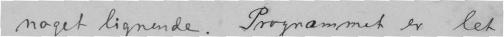noget lignende. Programmet er let
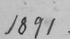1891
